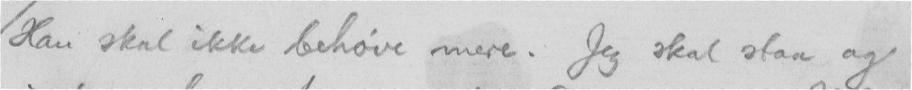Han skal ikke behøve mere. Jeg skal staa og
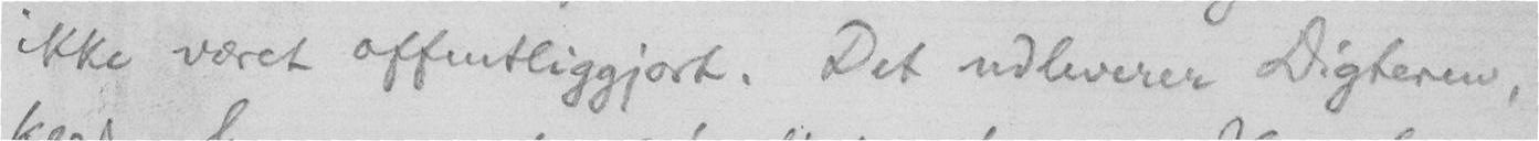ikke været offentliggjort. Det udleverer Digteren,
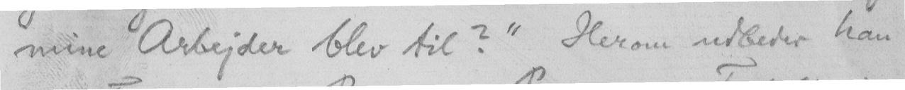mine Arbejder blev til?" Herom udbeder han
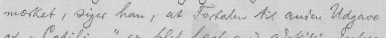mærket, siger han, at Fortalen til anden Udgave
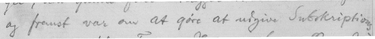og fremst var om at gøre at udgive Subskriptions-
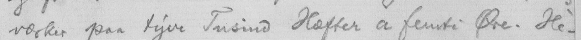værker paa tyve Tusind Hæfter a femti Øre. He-
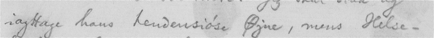iagttage hans tendensiøse Øjne, mens Helse-
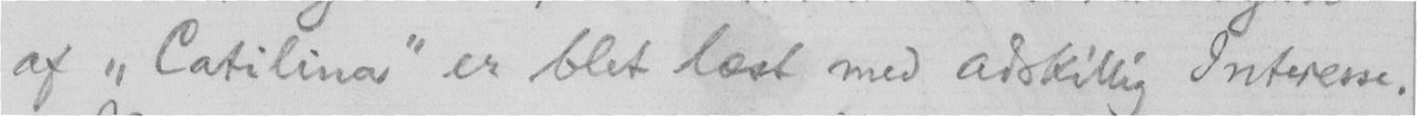af "Catilina" er blet læst med adskillig Interesse.
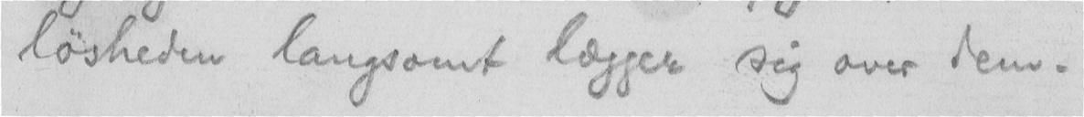løsheden langsomt lægger sig over dem.
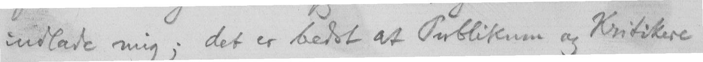indlade mig; det er bedst at Publikum og Kritikere
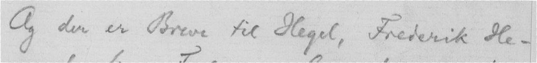Og der er Breve til Hegel, Frederik He-
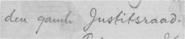den gamle Justitsraad.
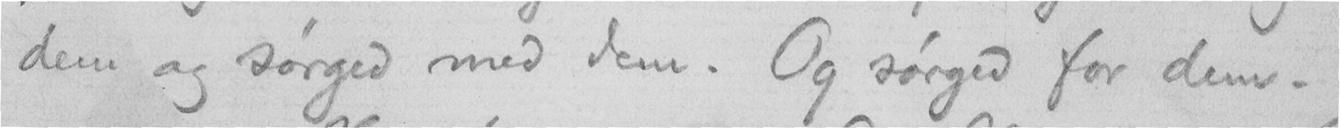dem og sørged med dem. Og sørged for dem.
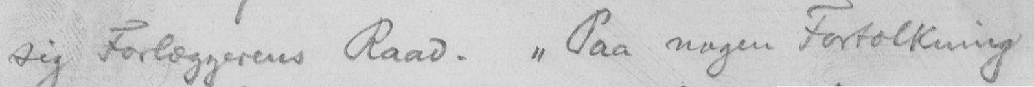sig Forlæggerens Raad. "Paa nogen Fortolkning
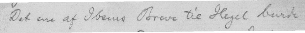Det ene af Ibsens Breve til Hegel burde
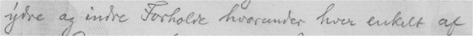ydre og indre Forholde hvorunder hver enkelt af
